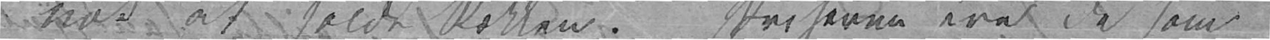nok at holde Rækken. Priserne ere de som
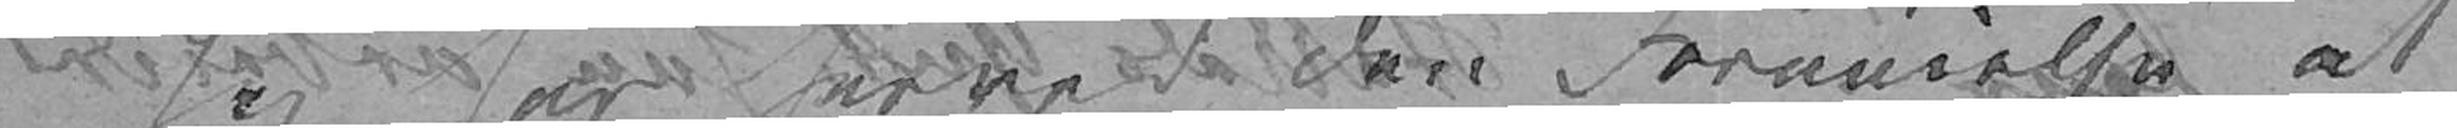Jeg har herved den Fornøielse at
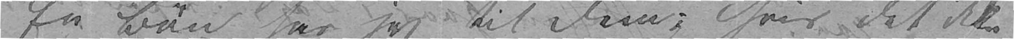En Bøn har jeg til Dem; hvis det ikke
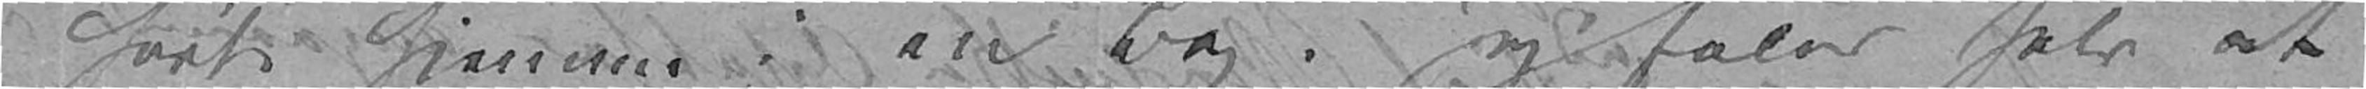hørte hjemme i en Bog. Jeg føler selv at
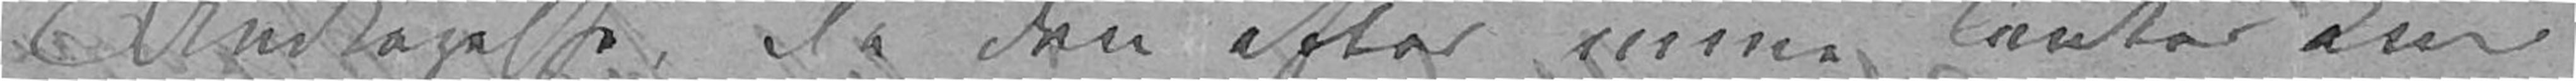Undtagelse, da den efter mine Tanker kun
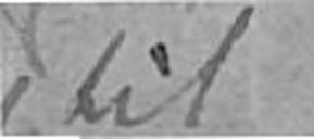til
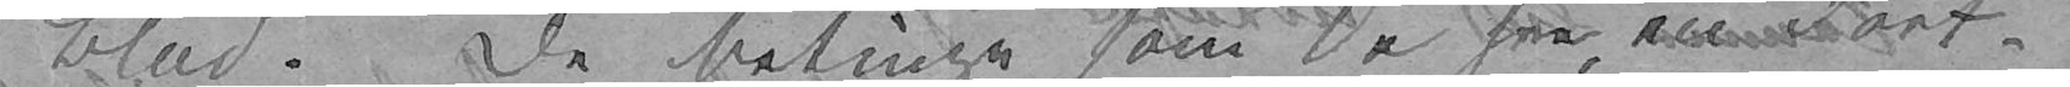Blad. De betinge som De see, en Fort¬
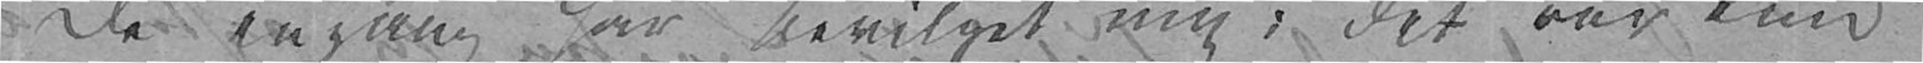De engang har bevilget mig; det var kun
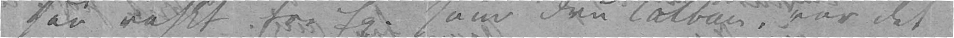saa raskt for Ex. som Fru Colban, var det
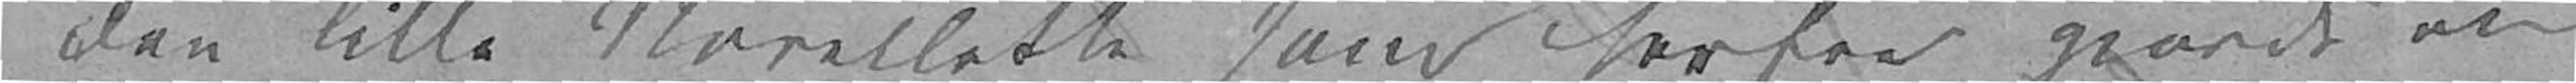den lille Novellette som herfra gjorde en
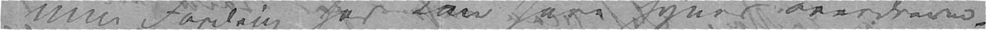min Fordring her kan have synes overdreven –
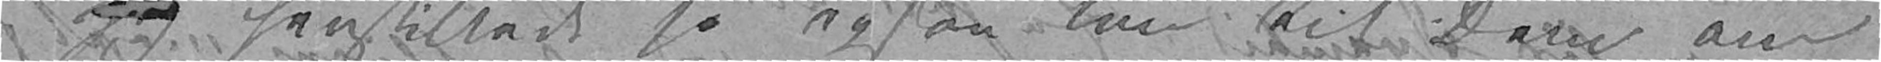jeg henstillede jo ogsaa kun til Dem om
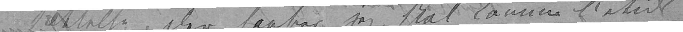sættelse der, haaber jeg, skal komme betids
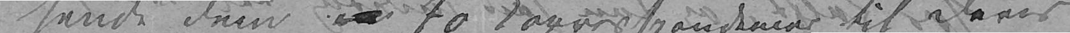sende Dem <gap /></del> to Korresspondencer til Deres
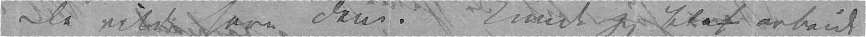De vilde have den. Kunde jeg blot arbeide
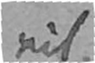vil
